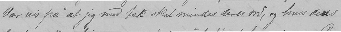Vær vis på at jeg med tak skal mindes deres ord, og hvis deres
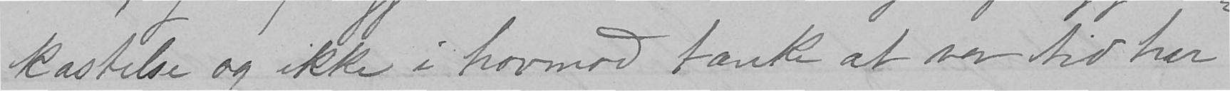kastelse og ikke i hovmod tænk at vor tid har
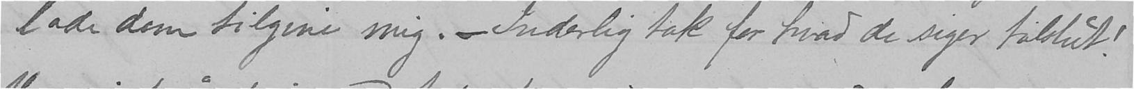lade dem tilgive mig. - Inderlig tak for hvad de siger tillslut!
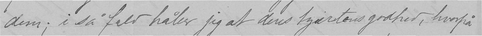dem; i så fald håber jeg at deres hjærtensgodhed, hvorpå
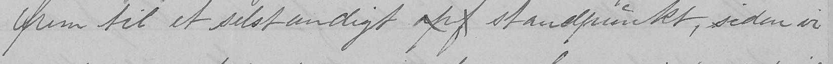frem til et selstændigt opf standpunkt, siden vi
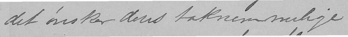det ønsker deres taknemmelige
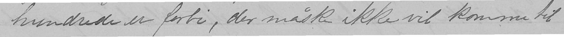hundrede er forbi, der måske ikke vil komme til
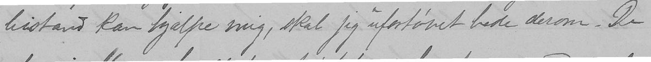bistand kan hjælpe mig, skal jeg ufortøvet bede derom. De
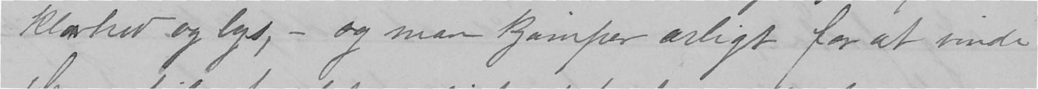klarhed og lys, - og man kjemper ærligt for at vinde
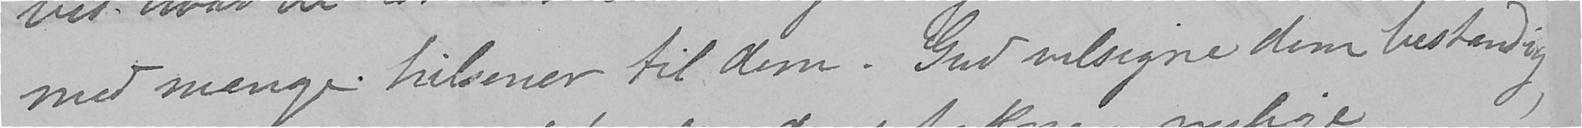med mange hilsener til dem. Gud velsigne dem bestandig,
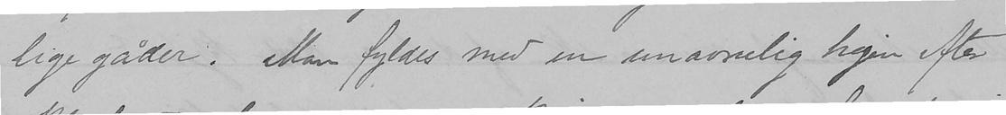lige gåder. Man fyldes med en unævnelig higen efter
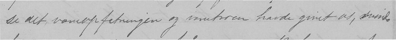se det vaneopfatningen og vanetroen havde givet os, svinde
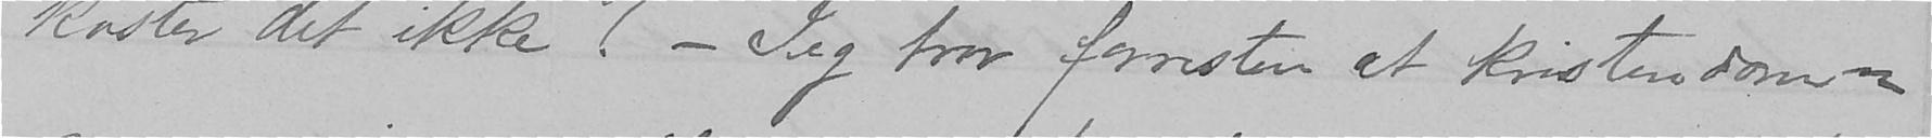koster det ikke! - Jeg tror forresten at Kristendom -
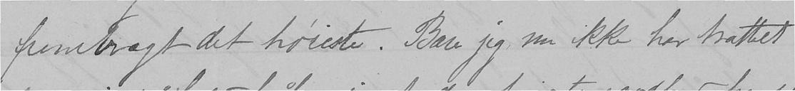frembragt det høieste. Bare jeg nu ikke har trættet
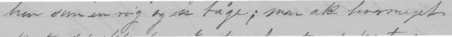hen som en røg og en tåge; men ak hvormeget
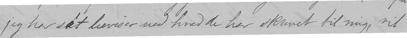jeg har sét beviser ved hvad de har skrevet til mig, vil
